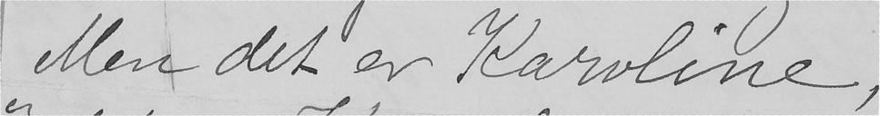"Men det er karoline,
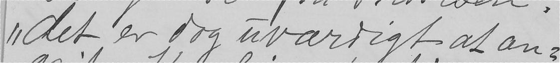"det er dog uværdigt at an-
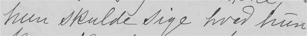hun skulde sige hvad hun
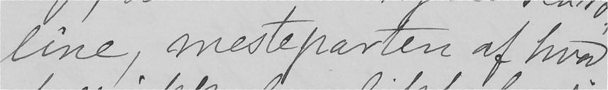line, mesteparten af hvad
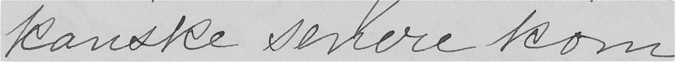kanske senere kom
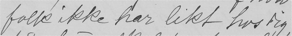folk ikke har likt hos dig,
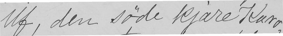Uf, den søde kjære Karo-
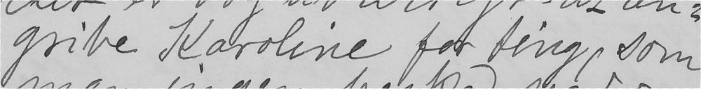gribe Karoline for ting, som
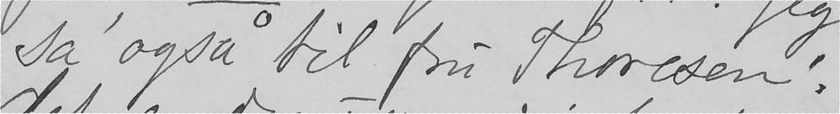sa' også til fru Thoresen:
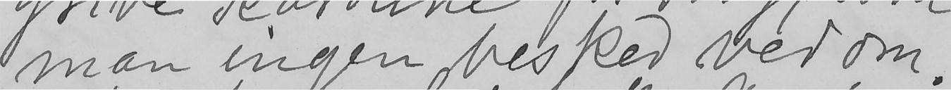man ingen besked ved om.
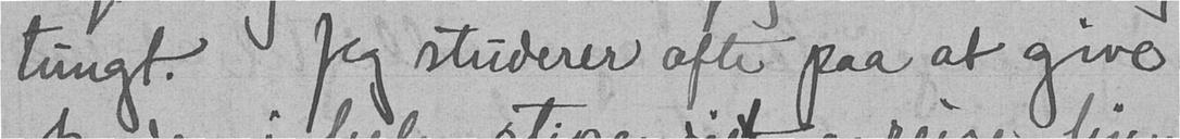tungt. Jeg studerer ofte paa at give
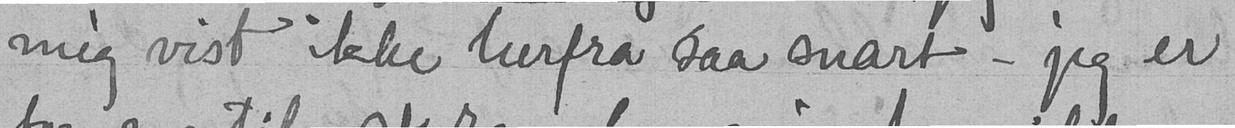mig vist ikke herfra saa snart - jeg er
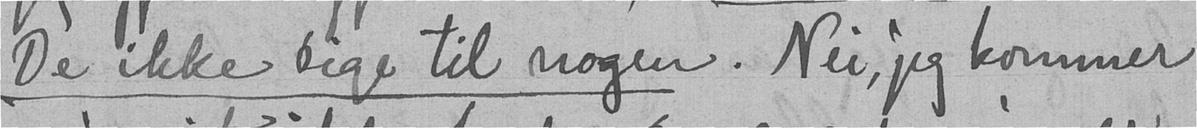De ikke sige til nogen. Nei, jeg kommer
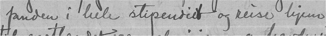fanden i hele stipendiet og reise hjem
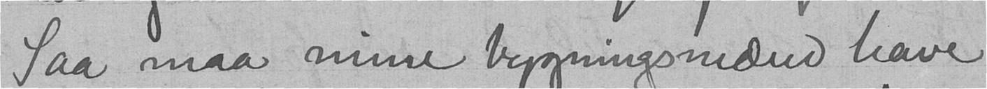Saa maa mine bygningsmænd have
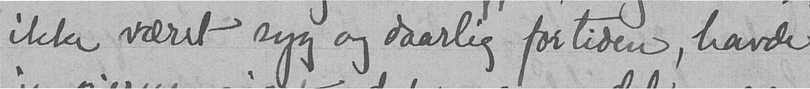ikke været syg og daarlig fortiden, havde
